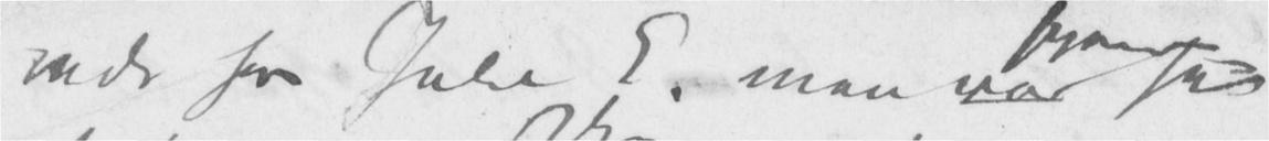inde hos Julie K. men var saa
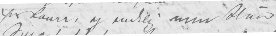hos Laura, og endelig min Stues
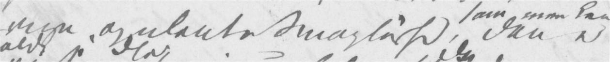nye, galante Smagløshed, den er
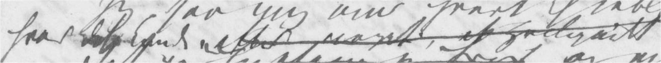hvad det kunde efter noget, et Hvilepunckt
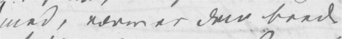med, værre er den baade
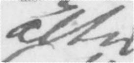altid
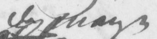de mange
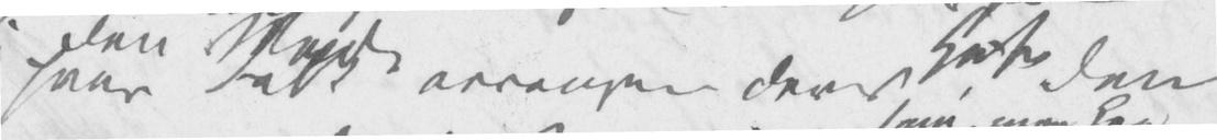hvor Folk arrangerer deres Huse, den
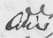<...>
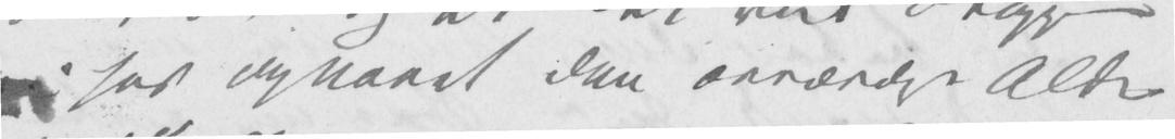nu har opnaaet den ærværdige Alder
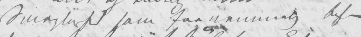Smagløshed som fornemmelig befor-
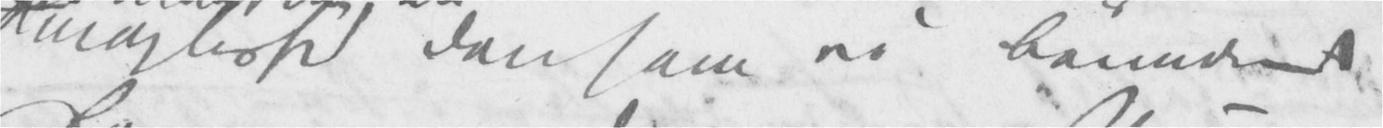Smagløshed den som vi beundrede
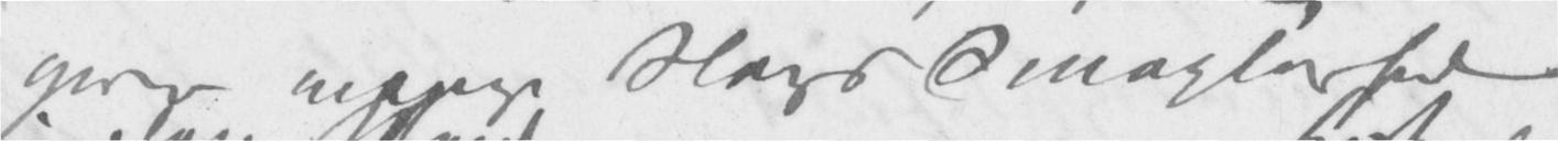gives mange Slags Smagløsheder
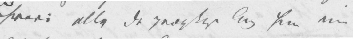hvori alle de prægtige Ting hun eier
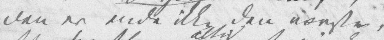den er enda ikke den værste,
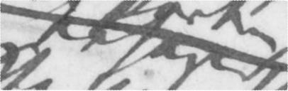<...>
